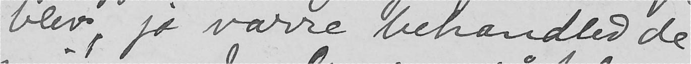blev, jo værre behandled de
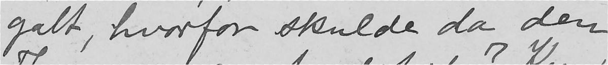galt, hvorfor skulde da den
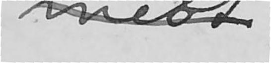mest
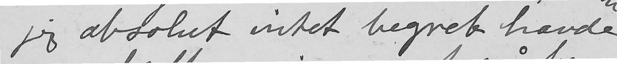jeg absolut intet begrep havde
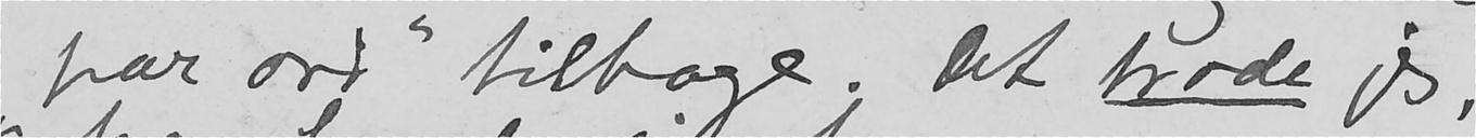"par ord" tilbage. Det trode jeg,
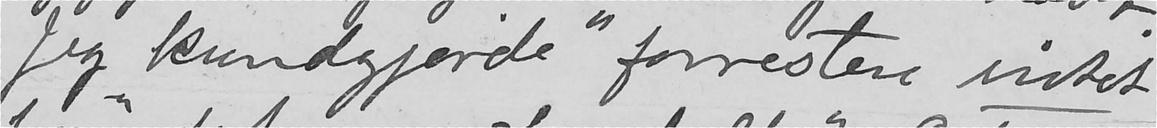Jeg "kundgjorde" forresten intet
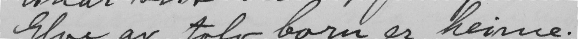Elve av tolv born er heime;
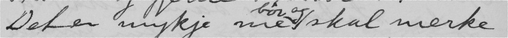Det er mykje me bör og skal merke
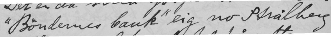"Böndernes bank" eig no Strålberg
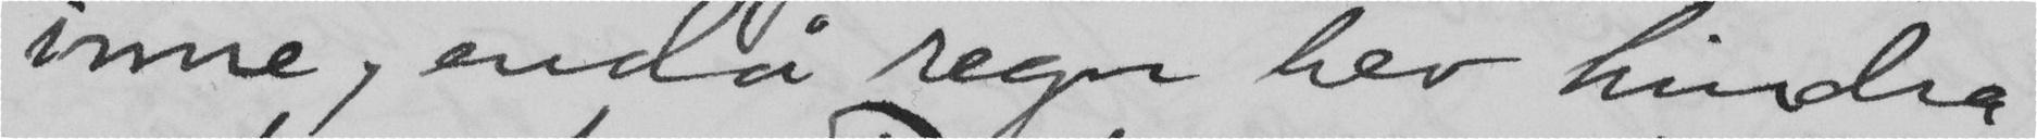inne, endå regn hev hindra
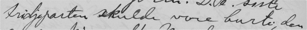tridjeparten skulde vore burte; den
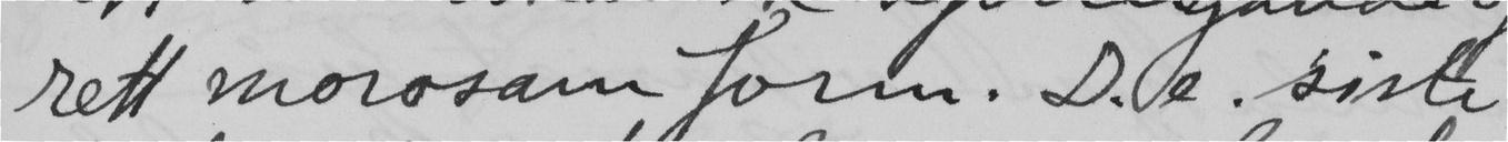rett morosam form. D.e. siste
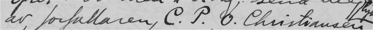av forfattaren, C.P.O. Christiansen
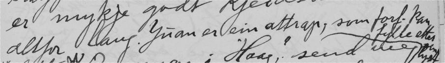altfor lang. Juan er ein attrap, som forf. kan fylte etter.
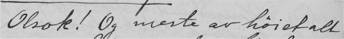Olsok! Og meste av höiet alt
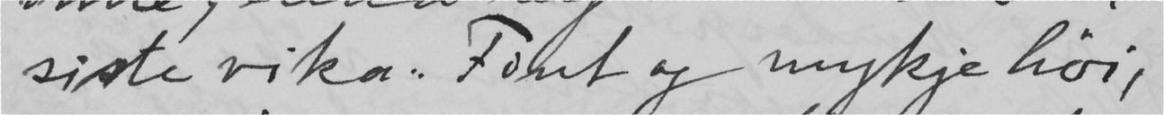siste vika. Fint og mykje höi,
